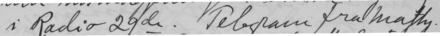i Radio 29de. Telegram fra Matty.
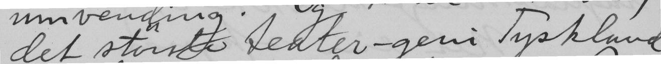det störste teater-geni Tyskland
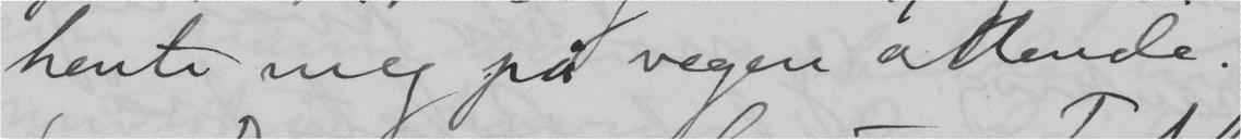hente meg på vegen attende.
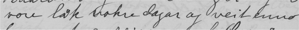vore låk nokre dagar og veit enno
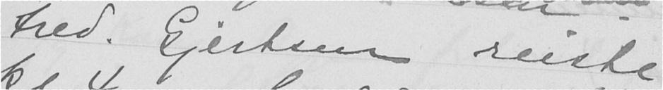Fred. Gjertsen reiste
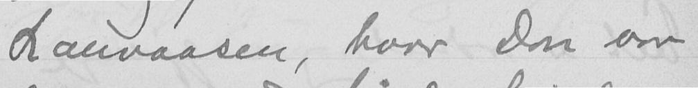Lauvaasen, hvor Don var
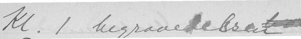Kl. 1 begravedelsen.
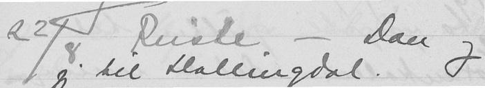22/8 Reiste - Don og
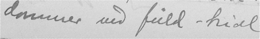dommer ved fuld-trial
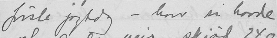første jagtdag - hvor vi havde
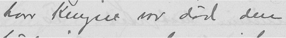hvor Kengiet var død den
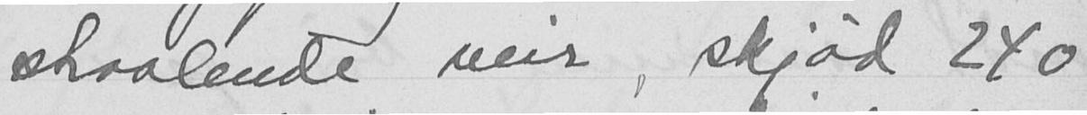straalende veir, skjød 240
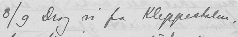8/9 Drog vi fra Kleppestølen,
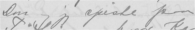Don og jeg spiste paa
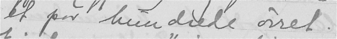et par hundrede ørret.
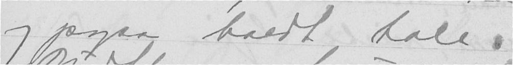og papa holdt tale.
